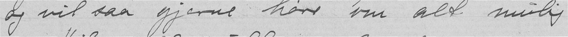og vil saa gjerne høre om alt mulig
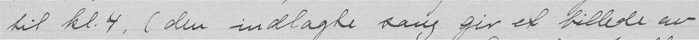til kl. 4, (den indlagte sang gir et billede av
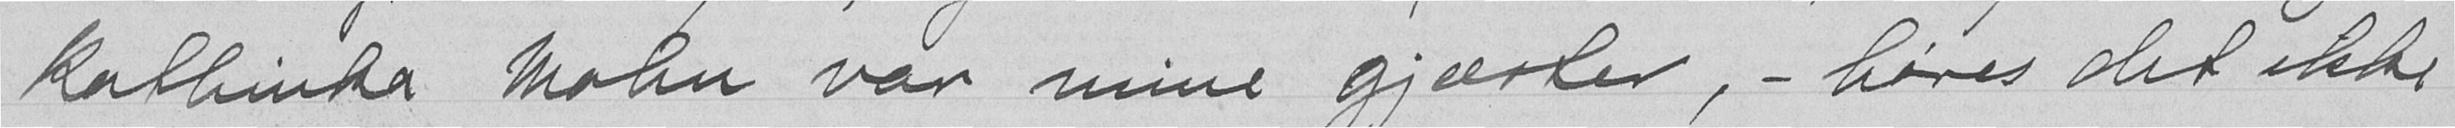Kathinka Molm var mine gjæster, - høres det ikke
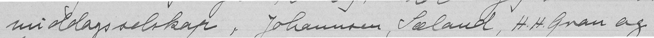middagsrelskap, Johannsen, Sæland, H.H. Gran og
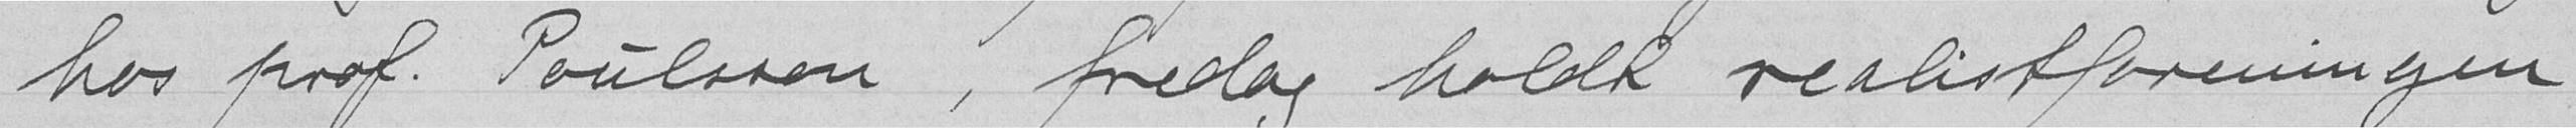hos prof. Poulssen, fredag holdt realistforeningen
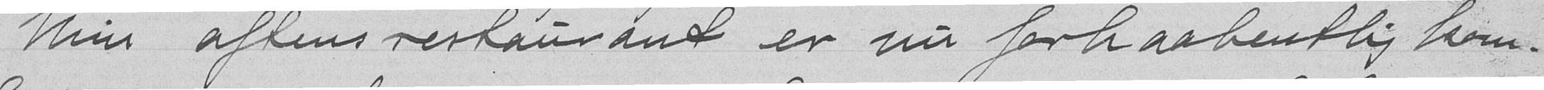Min aftensrestaurant er nu forhaabentlig kom-
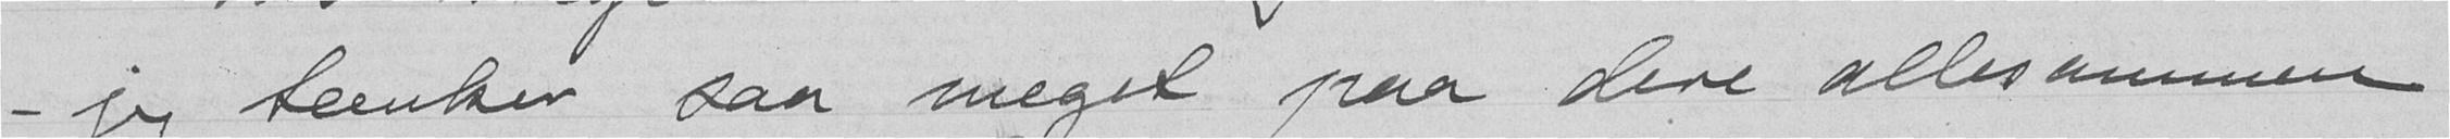jeg tænker saa meget paa dere allesammen
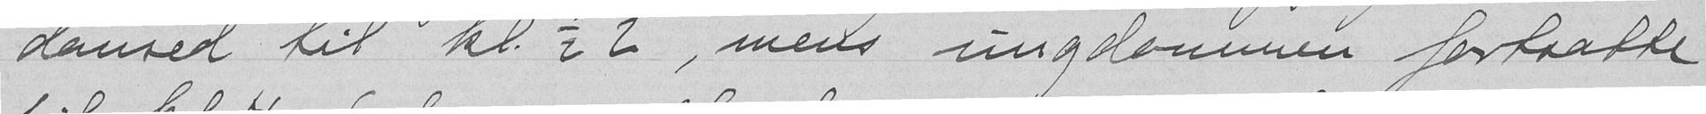dansed til kl. 22, mens ungdommen fortsatte
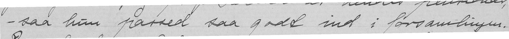- saa hun passed saa godt ind i forsamlingen.
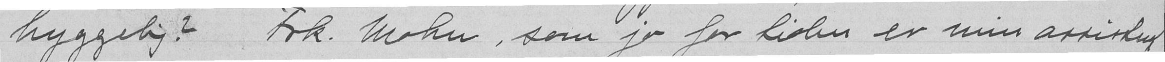hyggelig? Frk. Mohn, som jo for tiden er min assistent
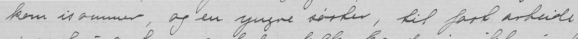kom isommer, og en yngre sørter, til fort arbeide
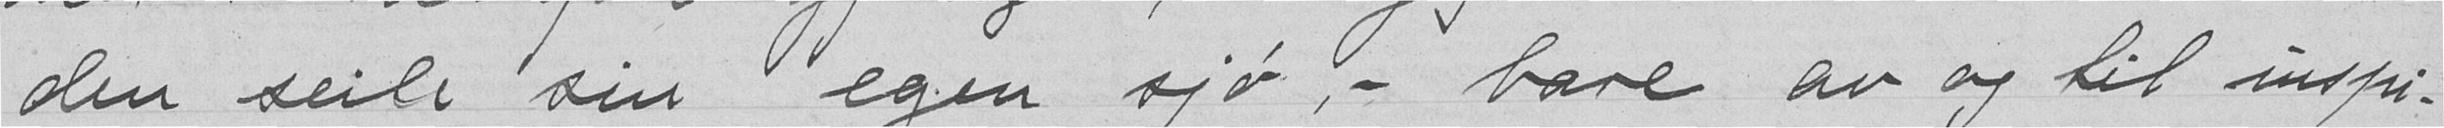den seile sin egen sjø ,- bare av og til inspi-
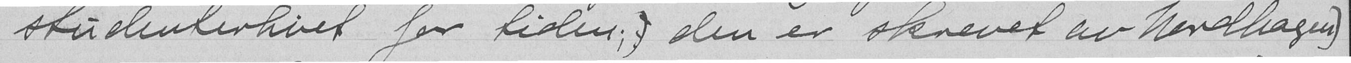studenterhvet for tiden; ) den er skrevet en Nordhagen)
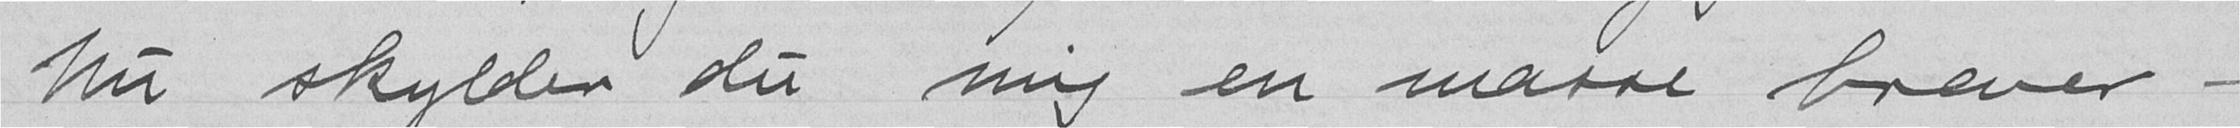nu skylder du ni en masse brever-
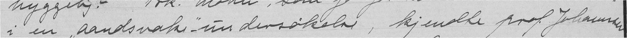i en "aandsværks"-undersøkelse, kjendte prof Johannes
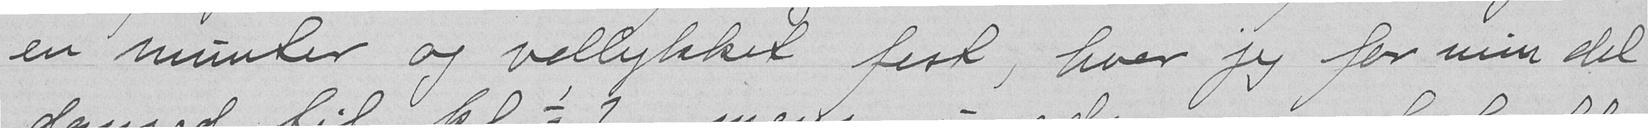en munter og vellykket fest, hvor jeg for min del
